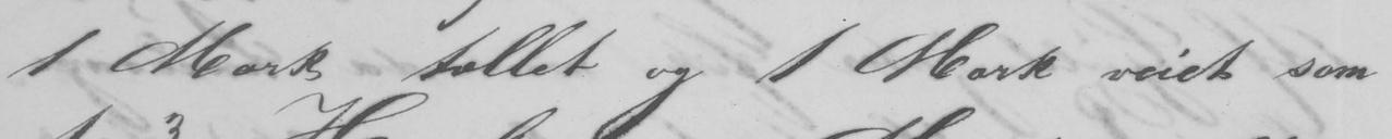1 Mark tællet og 1 Mark veiet som
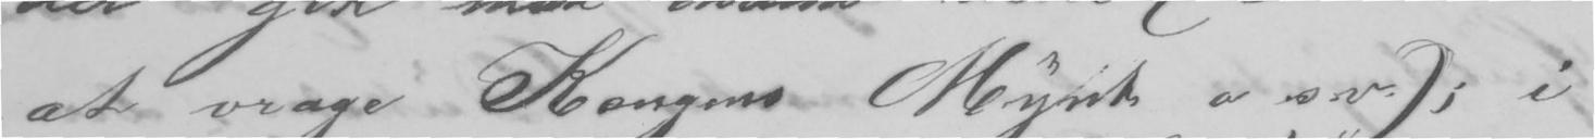at vrage Kongens Mynt osv.); i
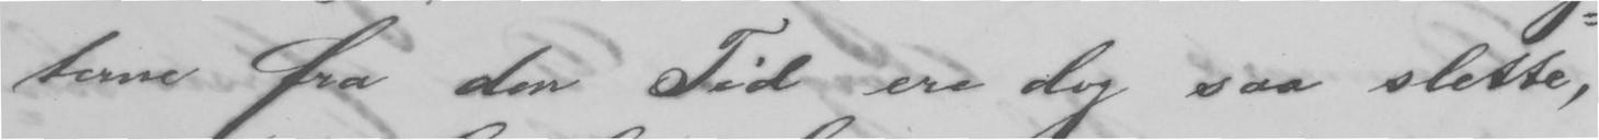terne fra den Tid er dog saa slette,
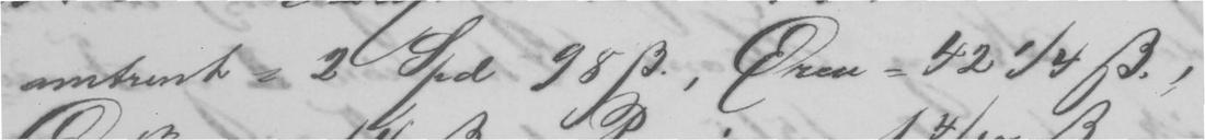omtrent = 2 Spd 98 ẞ., Øren = 42 ¼ ẞ.,
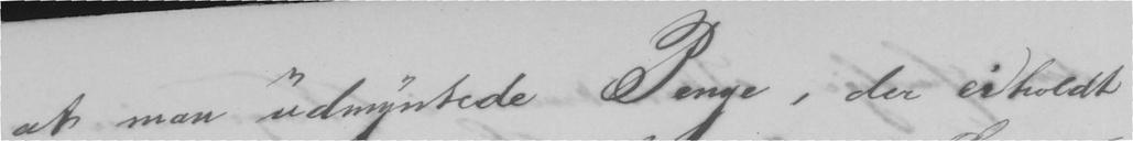at man udmyntede Penge, der ei holdt
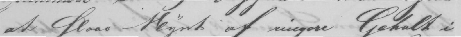at Slaa Mynt af ringen Gehalt i
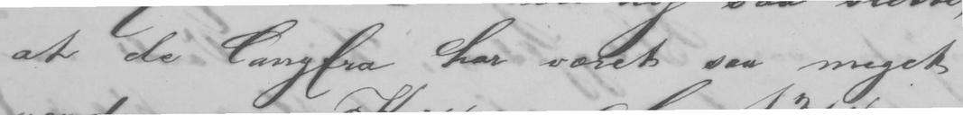at de langtfra har været saa meget
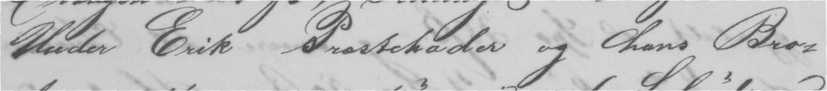Under Erik Prostekader og hans Bro-
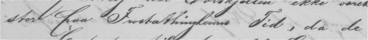stor paa Frostathinglovens Tid, da de
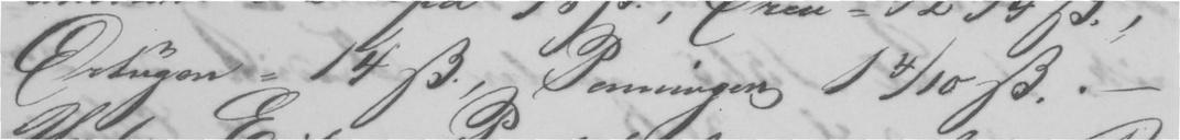Ørtugen= 14 ẞ., Penningen 1 ⁴⁄₁₀ ẞ. -
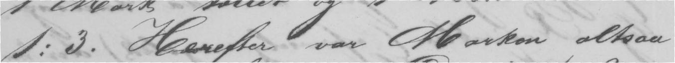1:3. Herefter var Marken altsaa
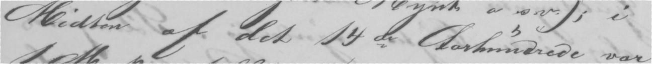Midten af det 14de Aarhundrede var
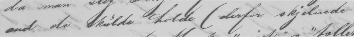end de Skulde holde (derfor skjelnede
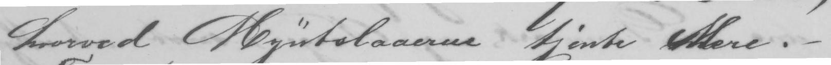hvorved Myntslaaerne tjente Mere. -
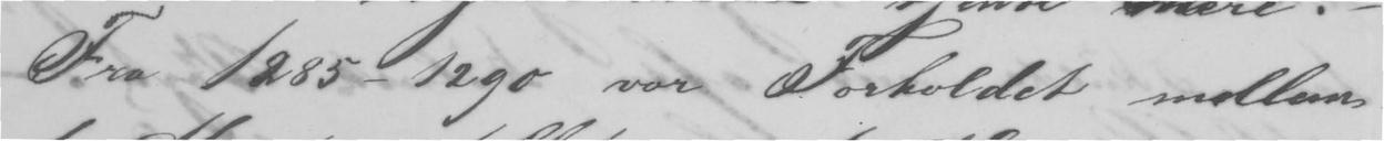Fra 1285-1290 var Forholdet mellem
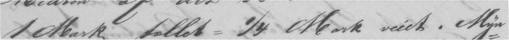1 Marks tællet = ¼ Mark veiet. Myn-
